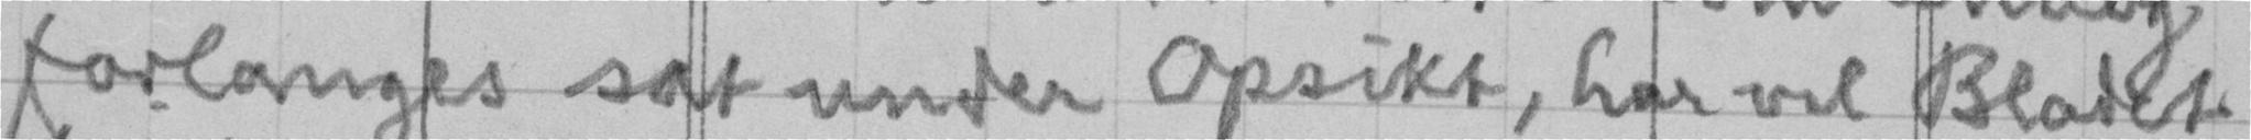forlanges sat under Opsikt, har vel Bladet
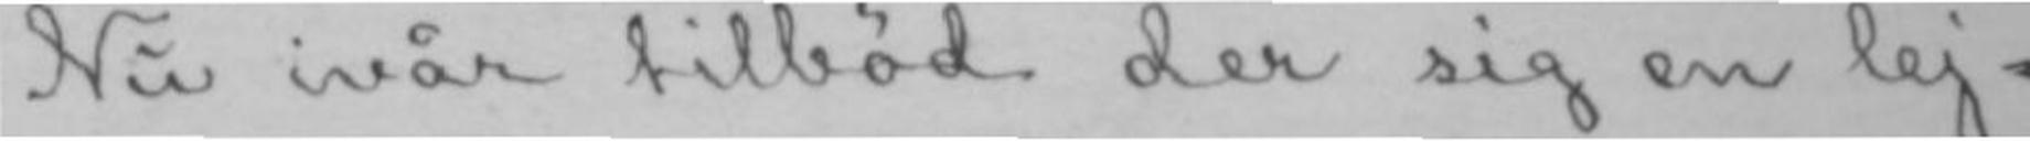Nu ivår tilbød der sig en lej-
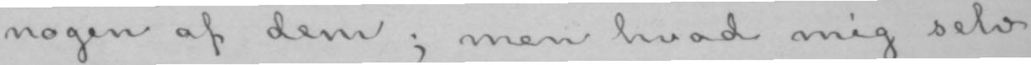nogen af dem; men hvad mig selv
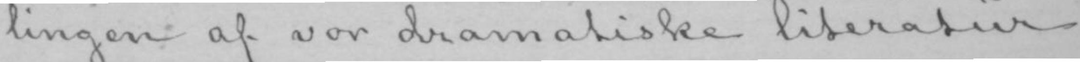lingen af vor dramatiske literatur,
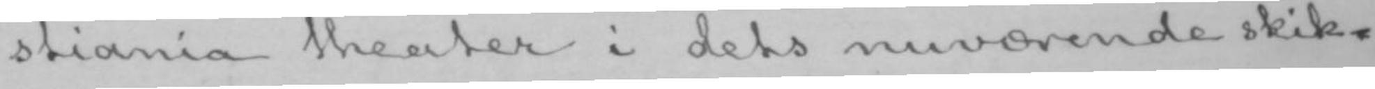stiania theater i dets nuværende skik-
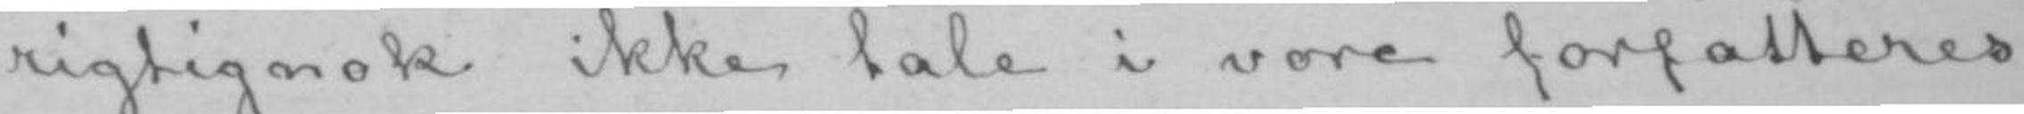rigtignok ikke tale i vore forfatteres
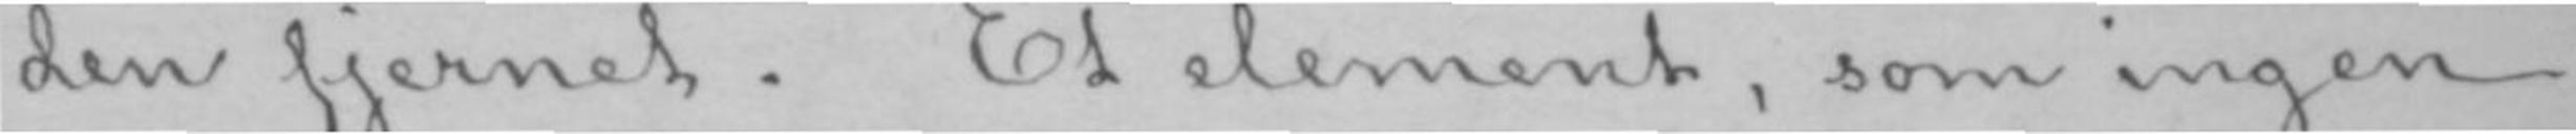den fjernet. Et element, som ingen
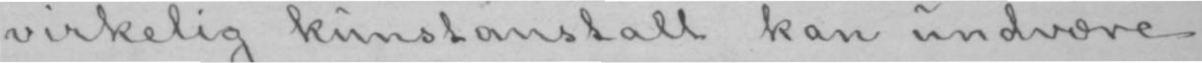virkelig kunstanstalt kan undvære,
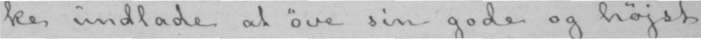ke undlade at øve sin gode og højst
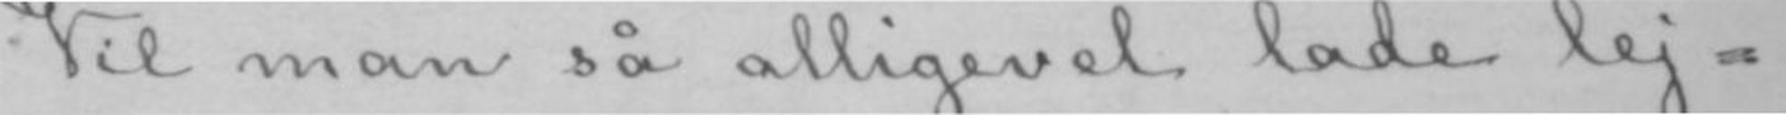Vil man så alligevel lade lej-
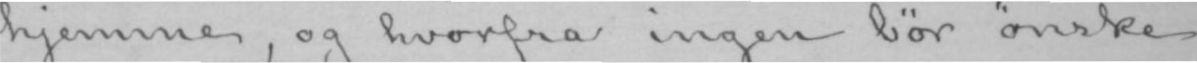hjemme, og hvorfra ingen bør ønske
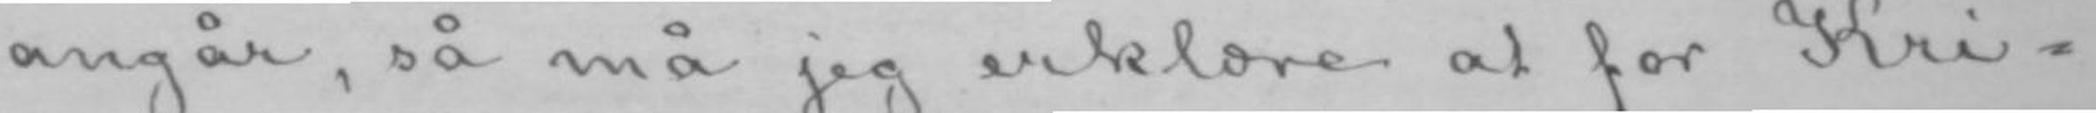angår, så må jeg erklære at for Kri-
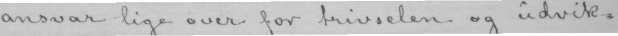ansvar lige over for trivselen og udvik-
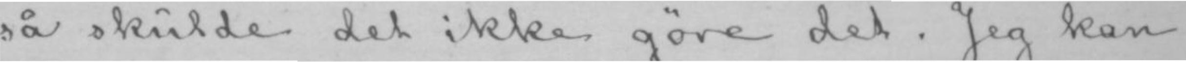så skulde det ikke gøre det. Jeg kan
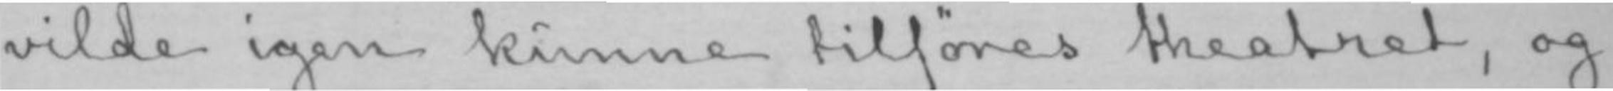vilde igen kunne tilføres theatret, og
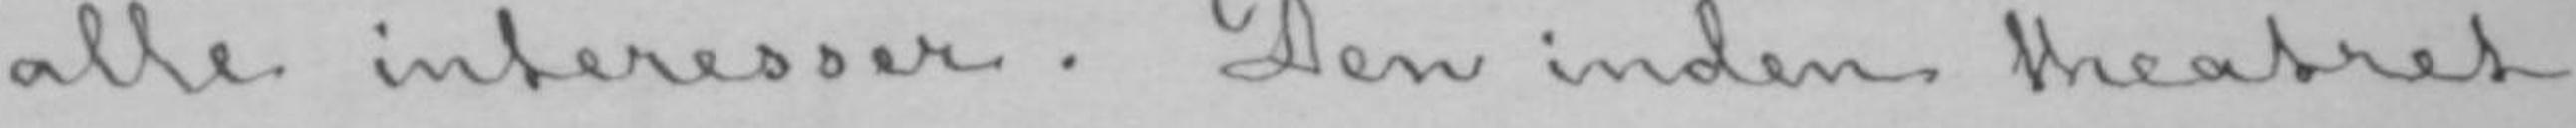alle interesser. Den inden theatret
